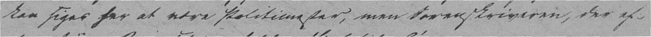kan siges her at være Politimester, men Sorenskriveren, der ef-
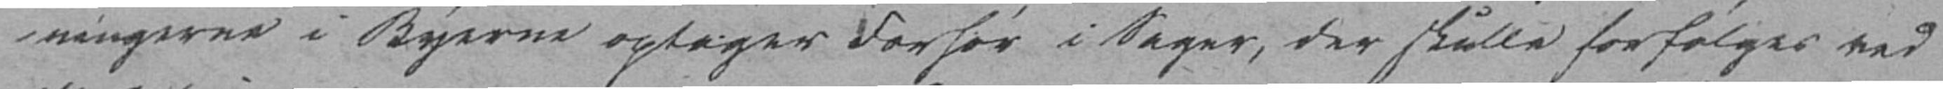ningerne i Byerne optager Forhør i Sager, der skulle forfølges ved
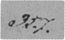357.
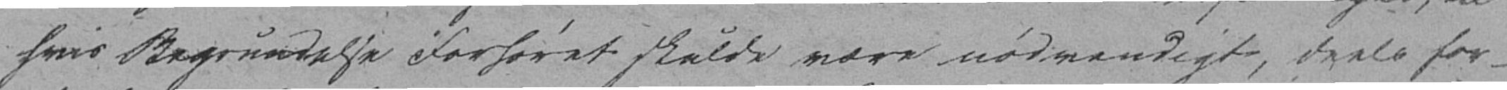hvis Begrundelse Forhøret skulde være nødvendigt, deels for-
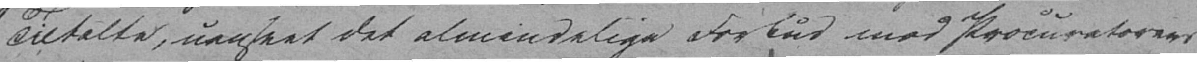Tiltalte, uanseet det almindelige Forbud mod Procuratorens
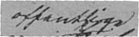offentlige
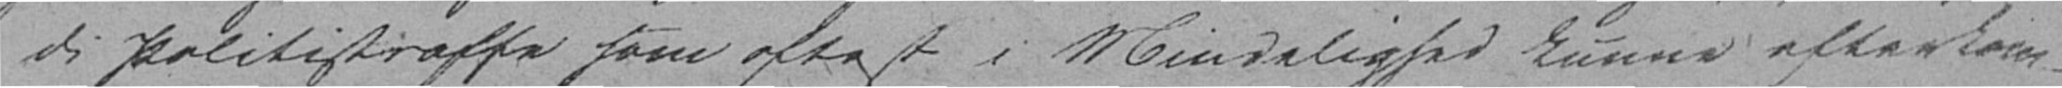di Politistraffe som oftest i Mindelighed kunne efterkom-
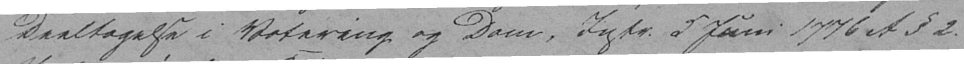Deeltagelse i Votering og Dom, Instr. 5 Juni 1776 et § 2.
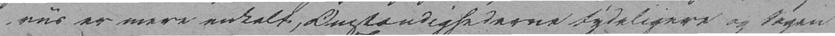viis er mere enkelt, Omstændighederne tydeligere og Sagen
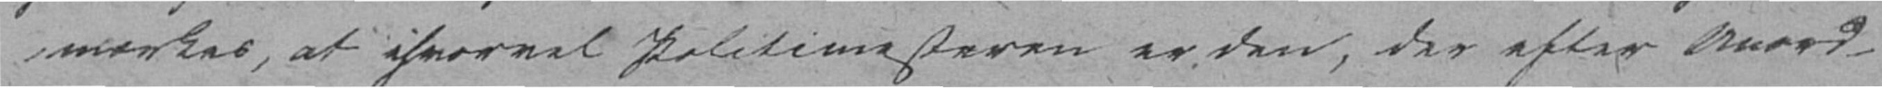mærkes, at ihvorvel Politimesteren er den, der efter Anord-
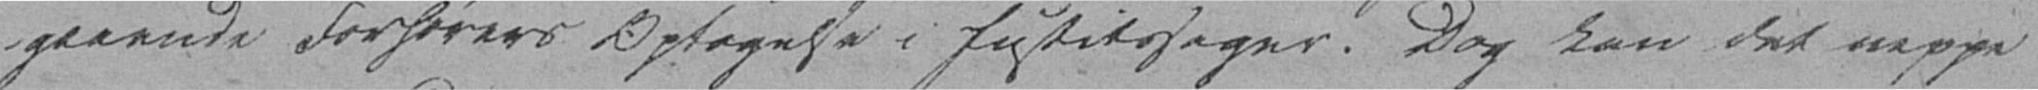gaaende Forhørers Optagelse i Justitssager. Dog kan det neppe
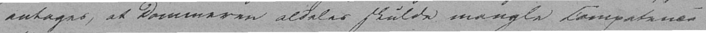antages, at Dommeren aldeles skulde mangle Competence
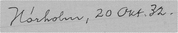Nørholm, 20 Okt. 32.
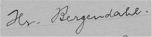Hr. Bergendahl.
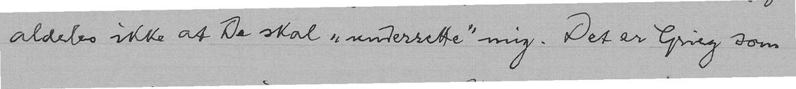aldeles ikke at De skal "underrette" mig. Det er Grieg som
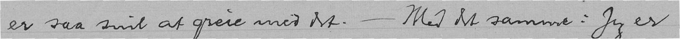er saa snil at greie med det. - Med det samme: Jeg er
